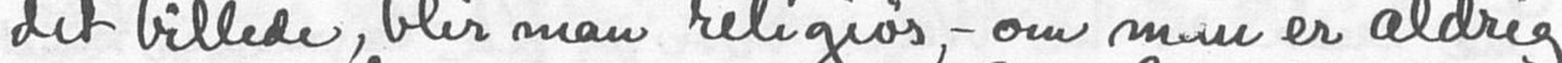det billede, blir man religiøs, - om man er aldrig
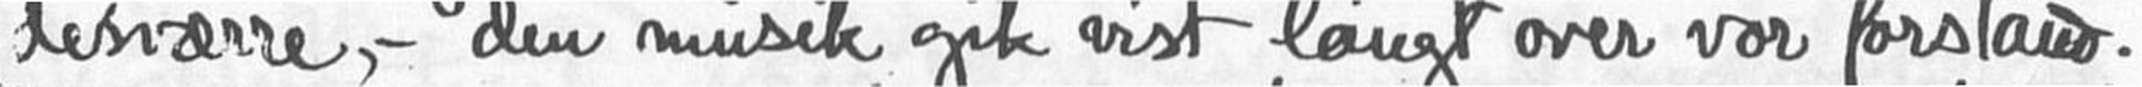desværre, - den musik gik vist langt over vor forstand.
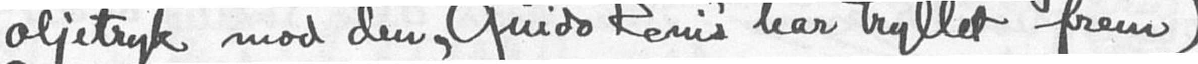oljetryk mod den, Guido Renis har tryllet frem).
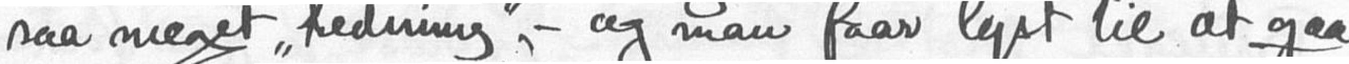saa meget "hedning", - og man faar lyst til at gaa
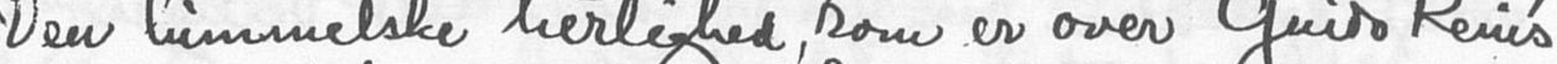Den himmelske herlighed, som er over Guido Renis
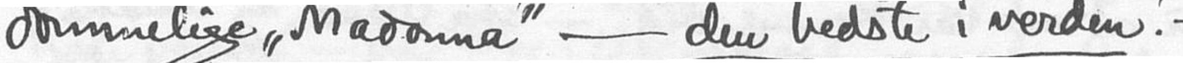dommelige "Madonna" - den bedste i verden ! -
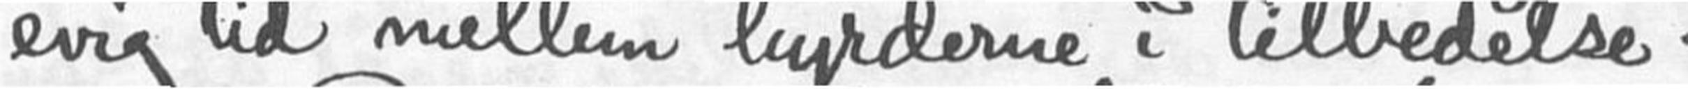evig tid mellem hyrderne i tilbedelse. -
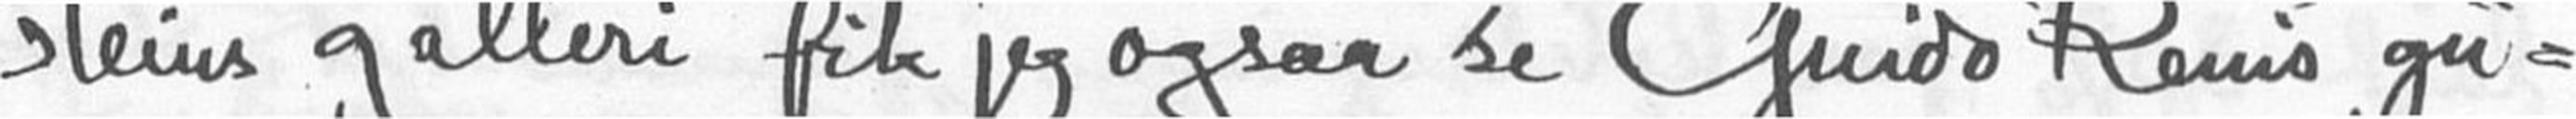steins galleri fik jeg ogsaa se Guido Reni's gu-
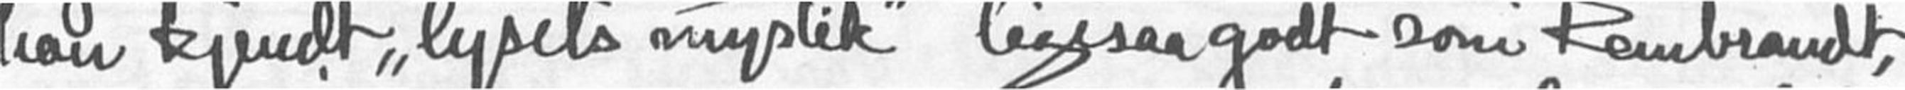han kjendt "lysets mystik" ligesaa godt som Rembrandt
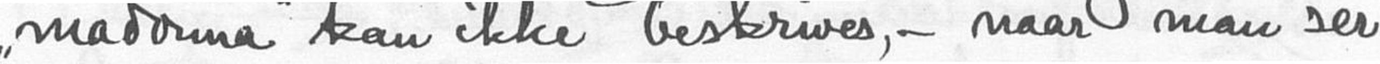"Madonna" kan ikke beskrives, - naar man ser
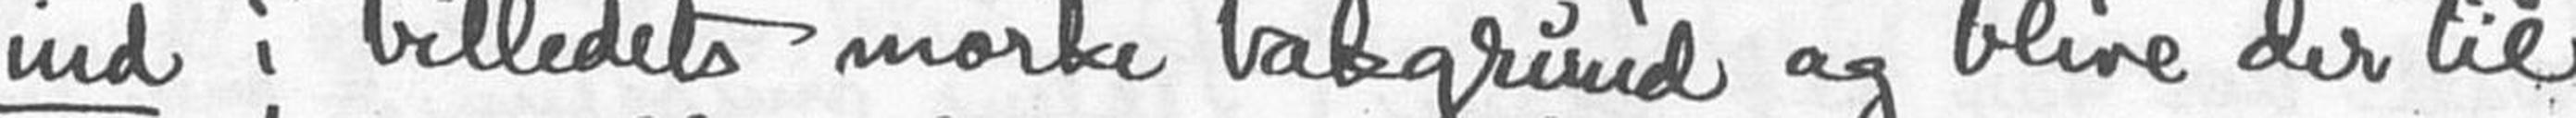ind i billedets mørke bakgrund og blive der til
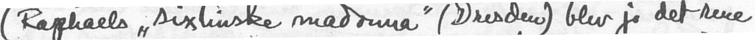(Raphaels "sixtinske madonna" (Dresden) blev jo det rene
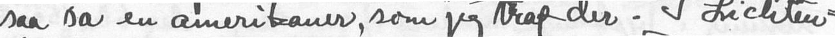saa sa en amerikaner, som jeg traf der. I Lichten-
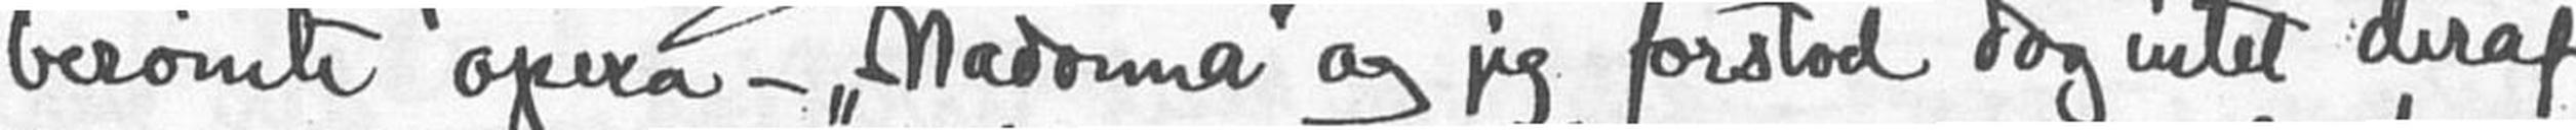berømte opera - "Madonna" og jeg forstod dog intet deraf
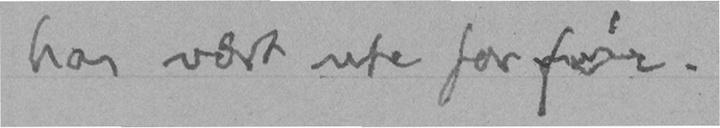har vært ute for før.
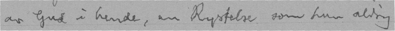av Gud i hende, en Rystelse som hun aldrig
